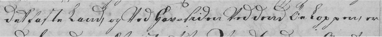det faste Land, og Ved Hav-siden Ved dend Øe Loppen, er
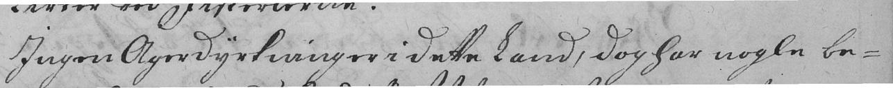Ingen Agerdyrkning er i dette Land, dog har nogle be-
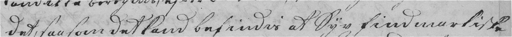det, saa som det kand befindis at Syv Findmarkiske
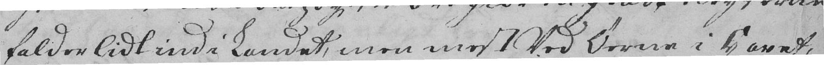falder lidt ind i Landet, men mest Ved Øerne i Havet,
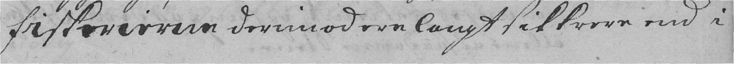Fiskerierne derimod ere langt sikkrere end i
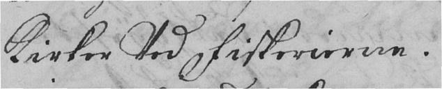Kirker Ved Fiskerierne.
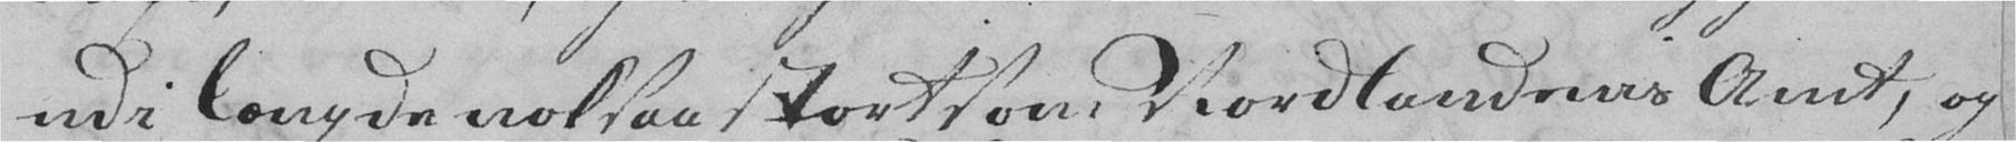udi længde noksaa stort som Nordlandenis Amt, og
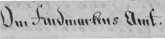Om Findmarkens Amt.
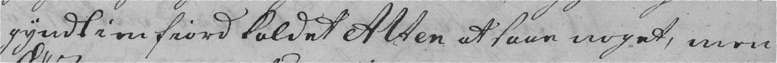gyndt i en fiord kaldet Alten at saae noget, men
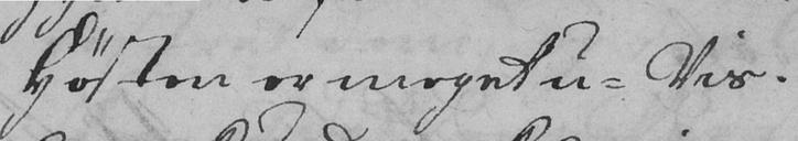Høsten er meget u-Vis.
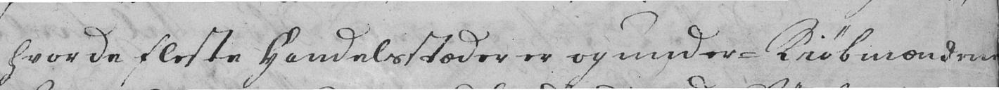hvor de fleste Handelsstæder er og under-Kiøbmændene
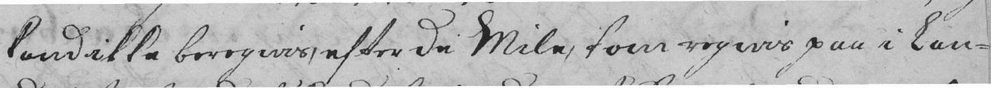kand ikke beregnis, efter de Mile, som regnis paa i Lan-
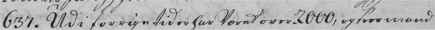637. Udi forrige tider har Været over 2000, og seer mand
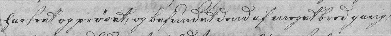har seet og prøvet, og befundet dend af meget bred gang,
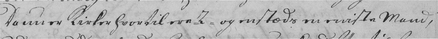Da nu er Kirker hvortil ere 2 og enstæds en eniste Mand,
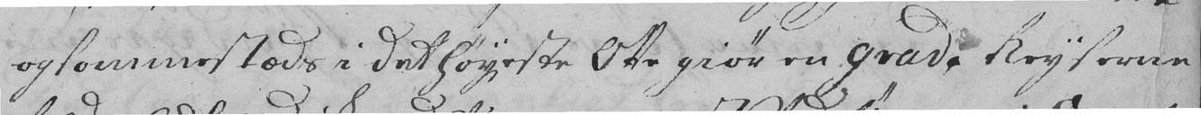og sommestæds i det høyeste Otte giør en grad. Reyserne
